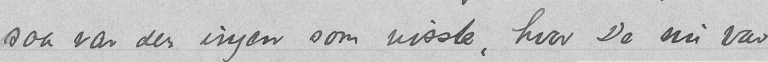saa var der ingen som visste, hvor De nu var
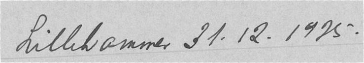Lillehammer 31.12.1925.
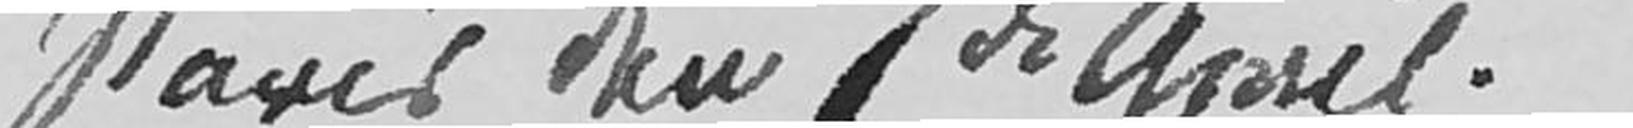Paris den 7de April.
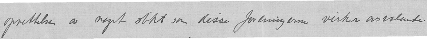oprettelsen av noget slikt som disse foreningerne virker avsvalende.
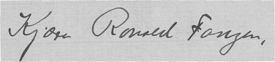Kjære Ronald Fangen,
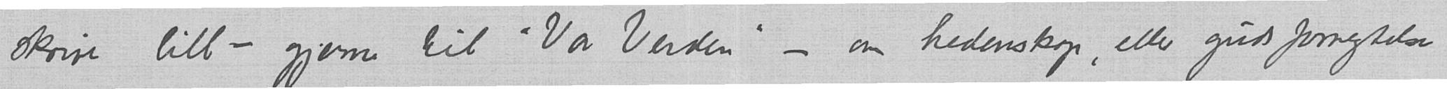skrive litt – gjerne til "Vor Verden" - om hedenskap, eller gudsfornegtelse
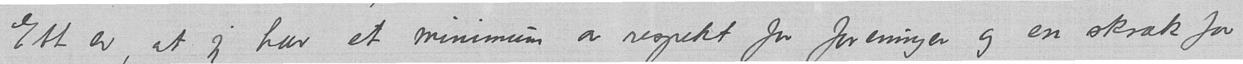Ett er, at jeg har et minimum av respekt for foreninger og en skræk for
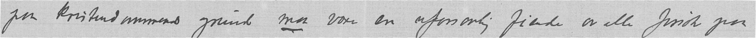paa kristiendommends grund maa være en uforsonlig fiende av alle forsøk paa
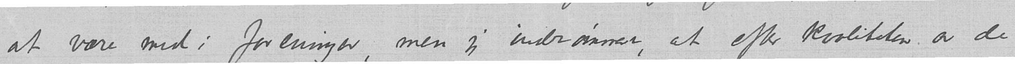at være med i foreninger, men jeg indrømmer, at efter kvaliteten av de
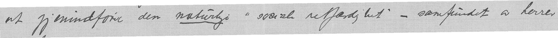at gjenindføre den naturlige «sosiale retfærdighet» – samfundet av herrer
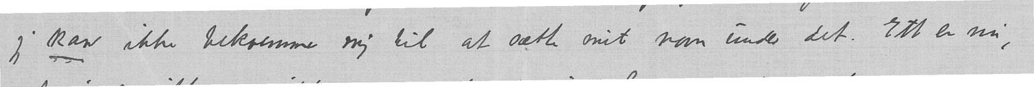jeg kan ikke bekvemme mig til at sætte mit navn under det. Ett er nu,
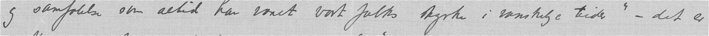og samfølelse som altid har været vort folks styrke i vanskelige tider" – det er
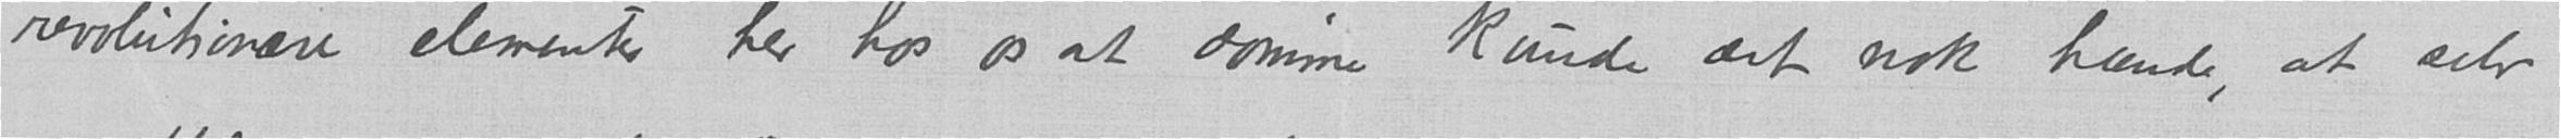revolutionere elementer her hos os at dømme kunde det nok hænde, at selv
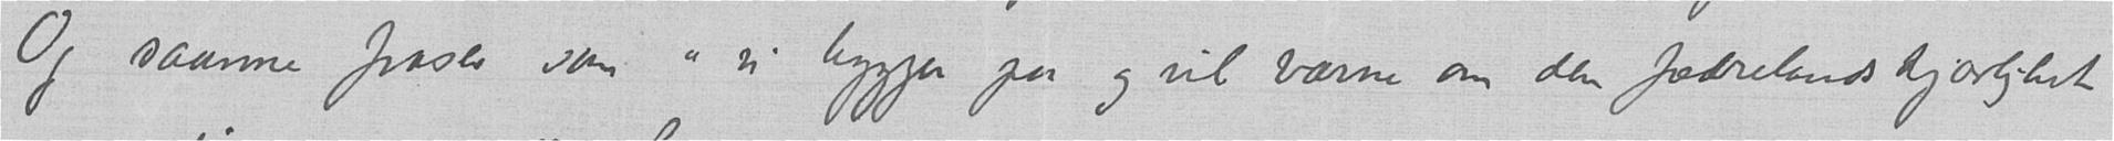Og saanne fraser som "vi bygger paa og vil værne om den fædrelandskjærlighet
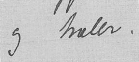og træler.
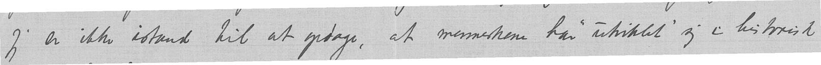jeg er ikke istand til at opdage, at menneskene har utviklet sig i historisk
